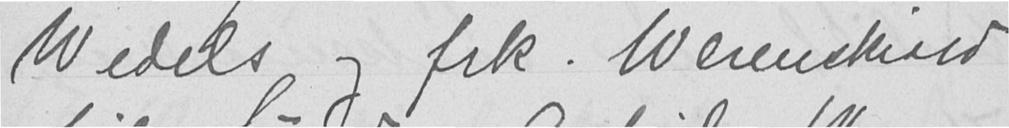Wedels og frk. Werenskiold
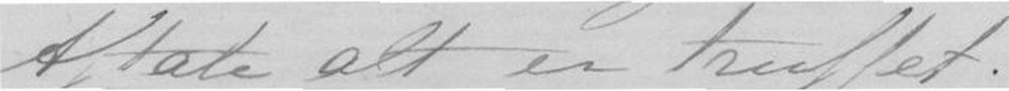Aftale alt er truffet.
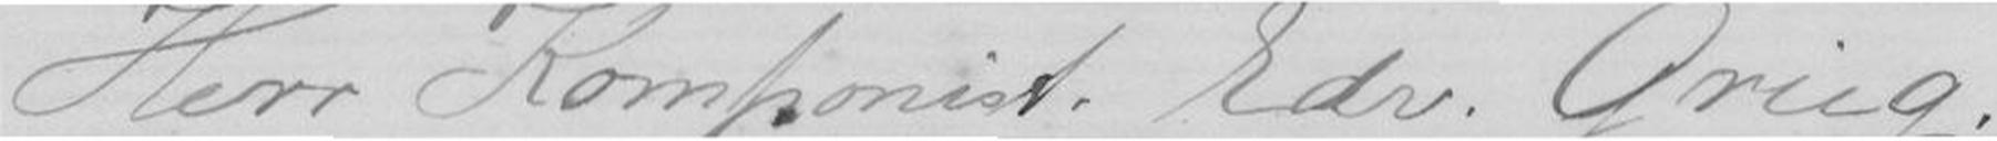Her Komponist Edv. Grieg.
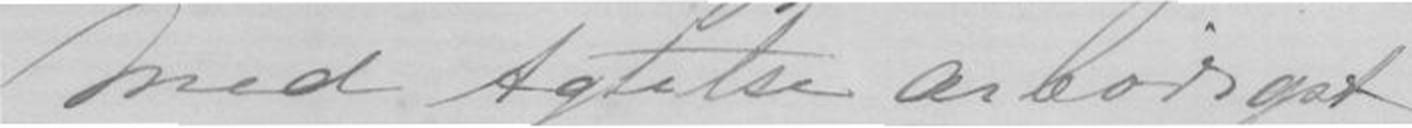Med Agtelse ærbødiget
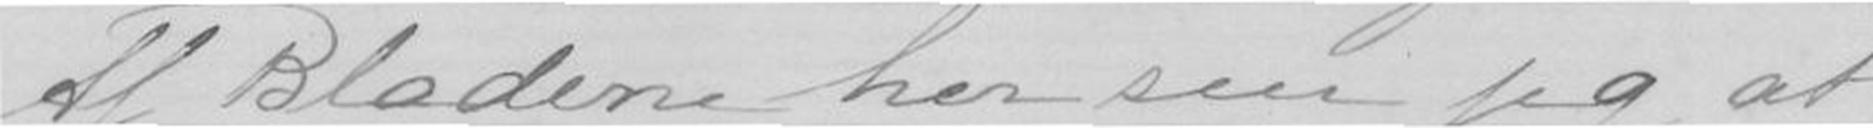Af Bladene her ser jeg at
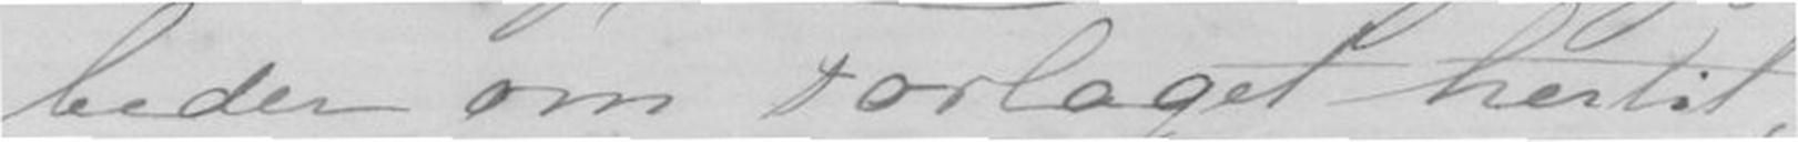beder om Forlaget hertil,
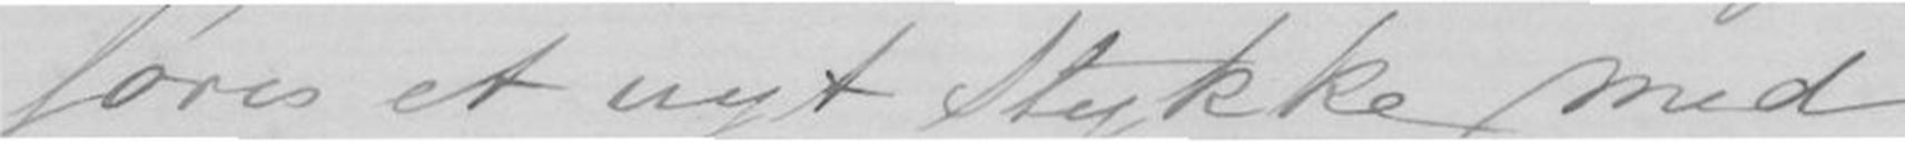føres et nyt Stykke med
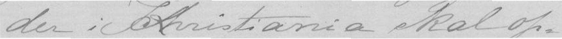der i Kristiania skal op-
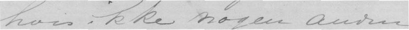hvis ikke nogen anden
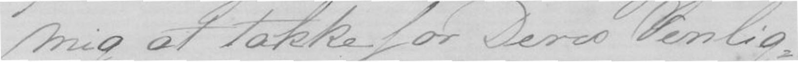mig at takke for Deres Venlig-
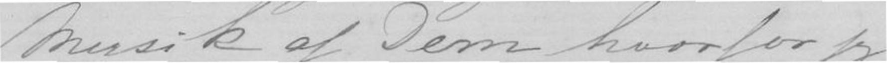musik af Dem hvorfor jeg
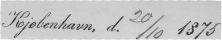Kjøbenhavn, d.20/10 1875
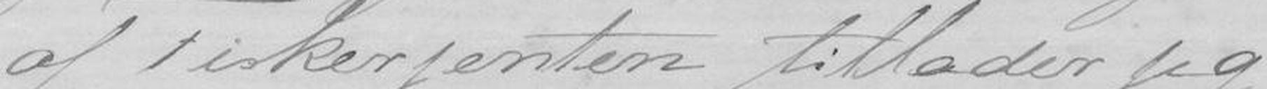af Fiskerjenten tillader jeg
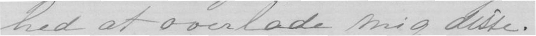hed at overlade mig disse.
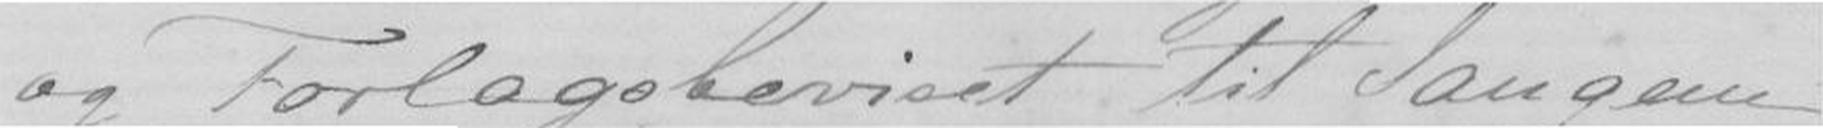og Forlagsbeviset til Sangene
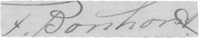F. Borchorst.
